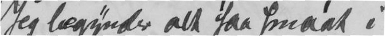Jeg begunder alt saa smaat i
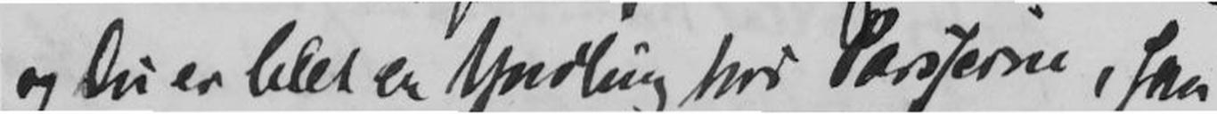og Du er blet en Yndling hos Pariserne, saa
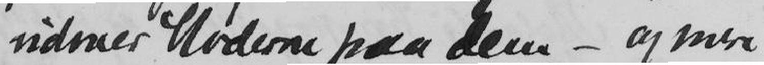udover Hoderne paa dem - og mere
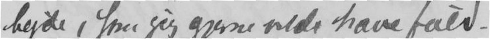bejde, som jeg gjerne vilde have fuld-
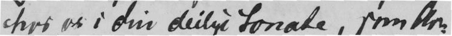hos os i din deilige Sonate, som Ar-
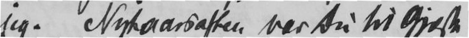jeg. Nytaarsaften var Du til Gjeste
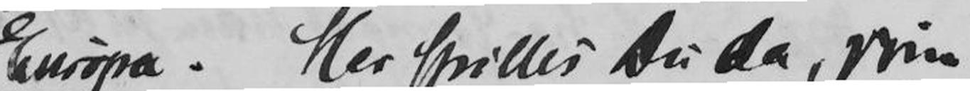Europa. Her spilles Du da, grin
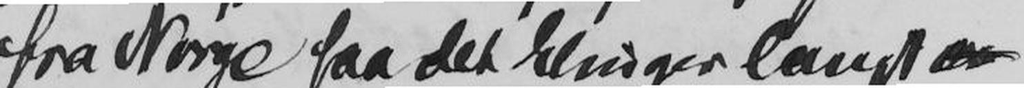fra Norge saa det klinger langt
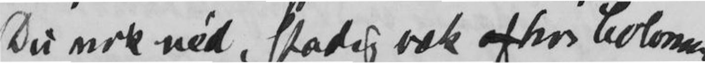Du nok véd, stadig væk af hos Colonne
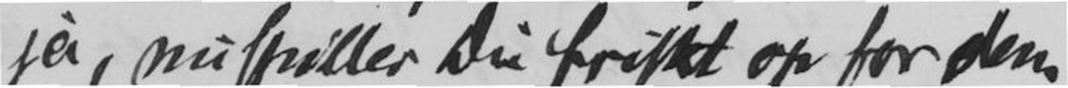ja, nu spiller Du friskt op for dem
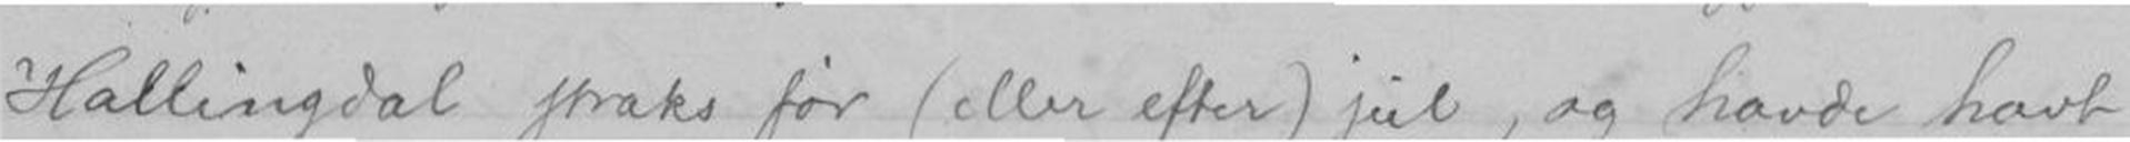Hallingdal straks før (eller efter) jul, og havde
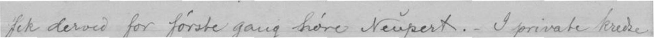fik derved for første gang høre Neupert. - I private kretser
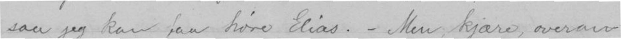saa jeg kan faa høre Elias. - Men kjære, overan-
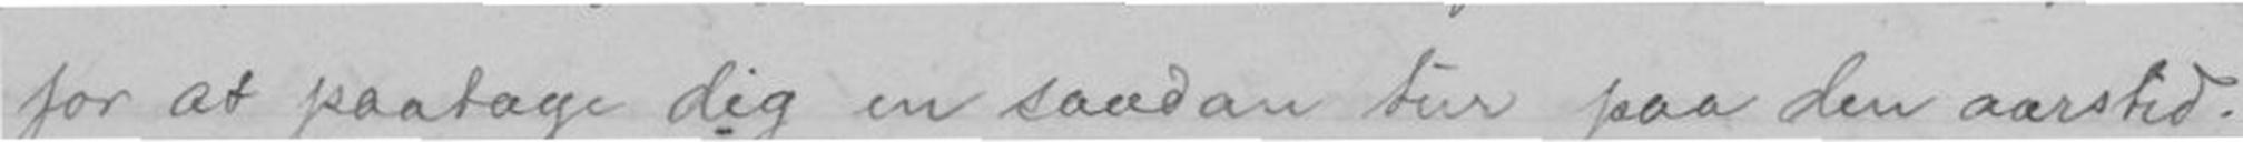for at paatage dig en saadan tur paa den aarstid.
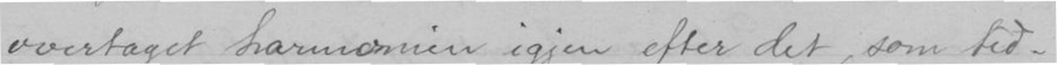overtaget harmonien igjen after det, som tid-
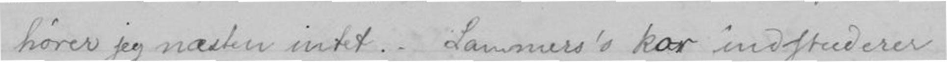hører jeg næsten intet. Lammers's kor indstuderer
